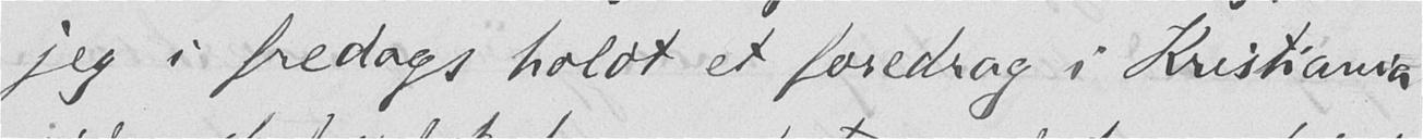jeg i fredags holdt et foredrag i Kristiania
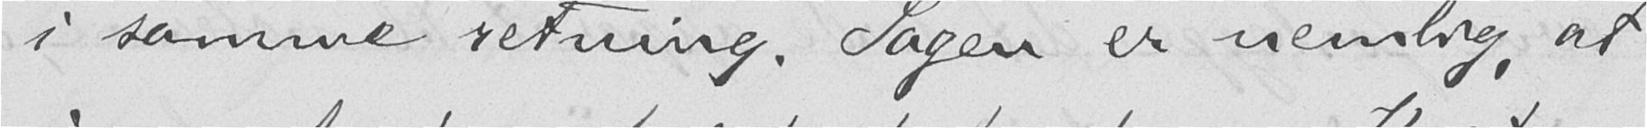i samme retning. Sagen er nemlig, at
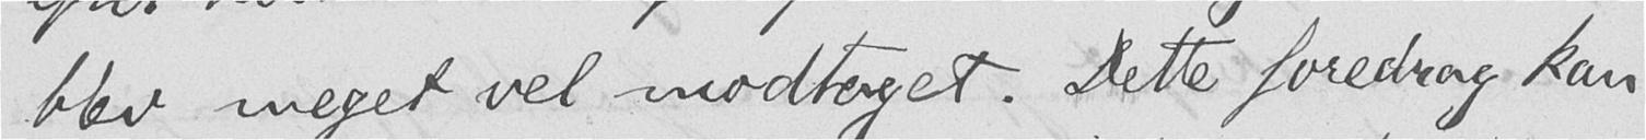blev meget vel modtaget. Dette foredrag kan
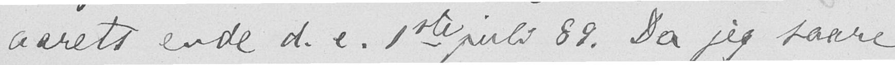aarets ende d. e. 1ste juli 89. Da jeg saare
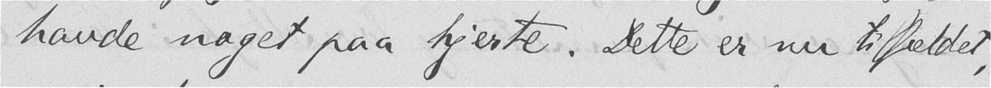havde noget paa hjerte. Dette er nu tilfældet,
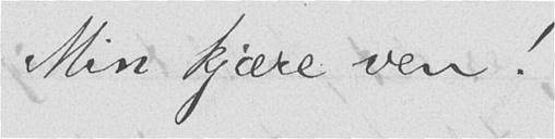Min kjære ven!
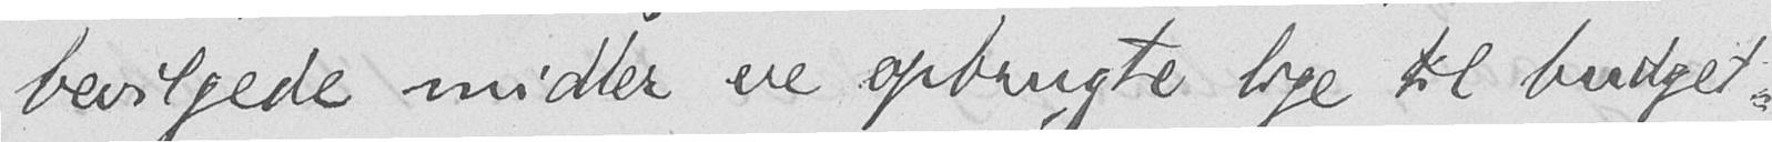bevilgede midler ere opbrugte lige til budget-
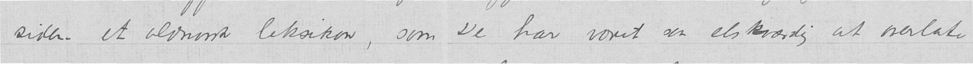siden et aldnorsk leksikon, som De har været saa elskværdig at overlate
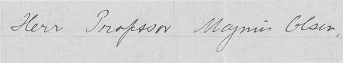Herr Professor Magnus Olsen,
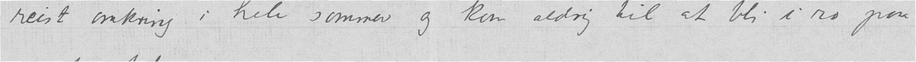reist omkring i hele sommer og kom aldrig til at bli i ro paa
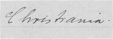Christiania.
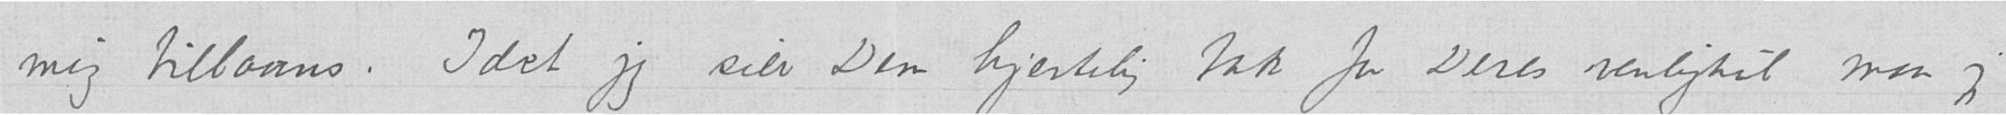mig tillaans. I det jeg sier Dem hjertelig tak for Deres venlighet maa jeg
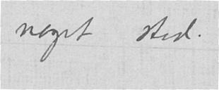noget sted.
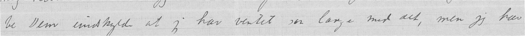be Dem undskylde at jeg har ventet saa længe med det, men jeg har
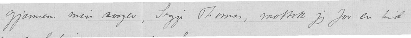gjennem min svoger, Sigge Thomas, mottok jeg for en tid
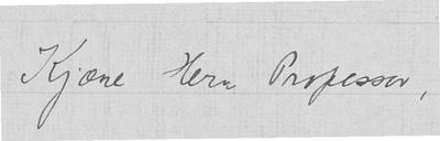Kjære Herr Professor,
